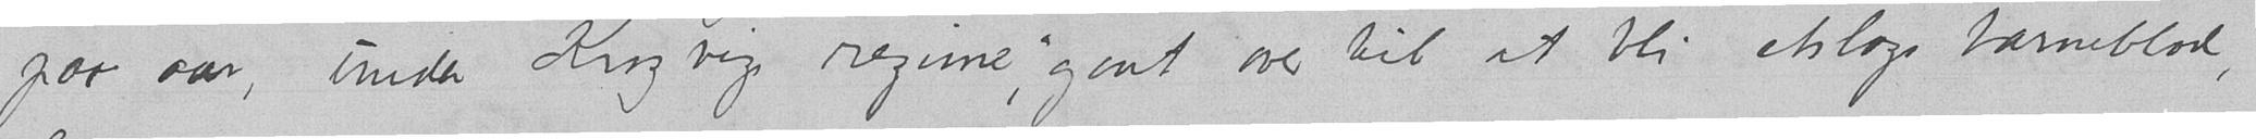par aar, under Krogvigs regime, gaat over til at bli etslags barneblad,
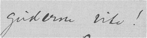guderne vite!
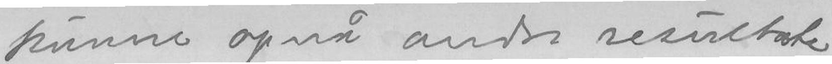kunne opnå andre resultater
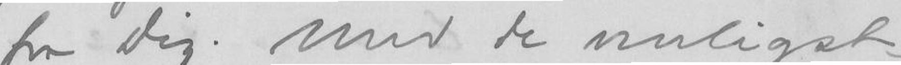fra dig. Med de venliget
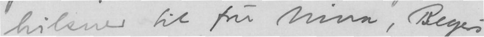hilsner til fru Nina, Beyers
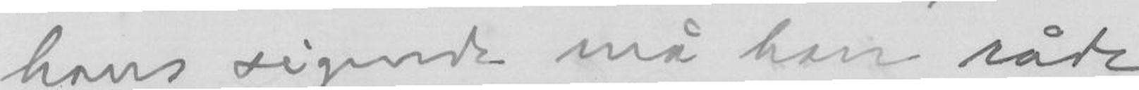hans sigende må han råde
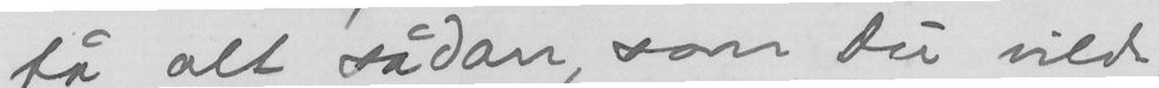få alt sådan, som Du vilde,
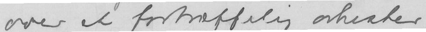over et fortræffelig orkester,
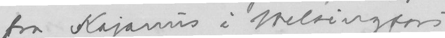fra Kajanus i Helsingfors
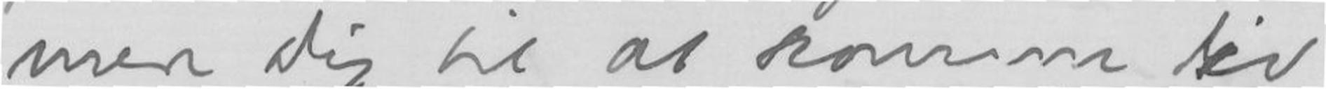mere dig til at komme hid
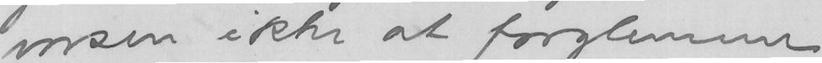vorsen ikke at forglemme.
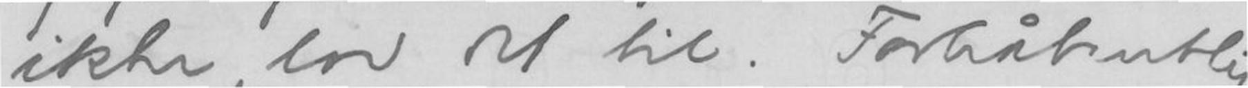ikke, lot det til. Forhåbentlig
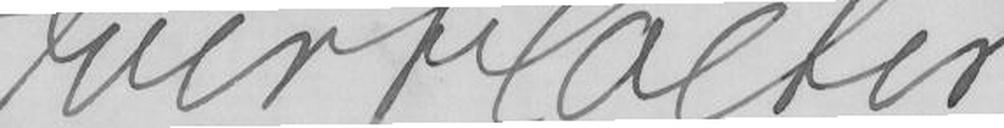Iver Holter
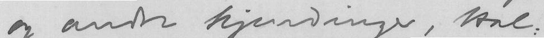og andre kjendinger. Hal-
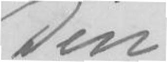Din
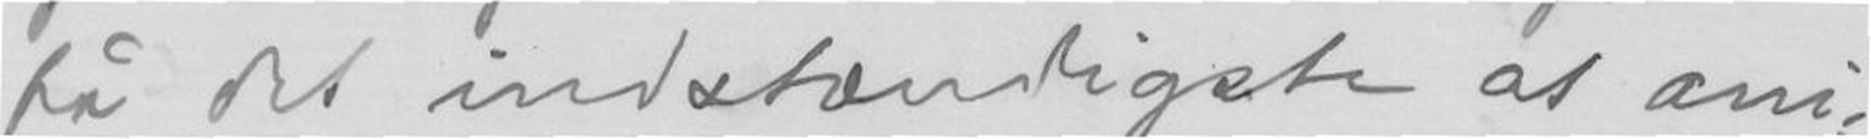på det indstændigste at ani-
